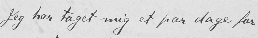Jeg har taget mig et par dage for
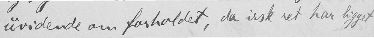uvidende om forholdet, da irsk ret har ligget
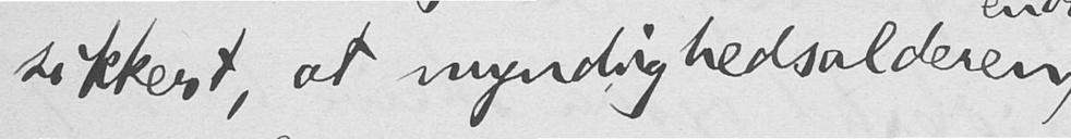sikkert at myndighedsalderen
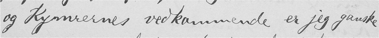og Kymrernes vedkommende er jeg ganske
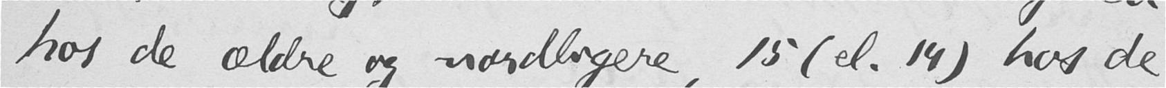hos de ældre og nordligere, 15 (el. 14) hos de
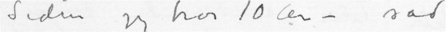Siden jeg tror 10 år – sad
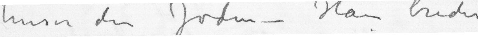husker den Jøden – han breder
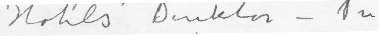Hotels Direktør – Du
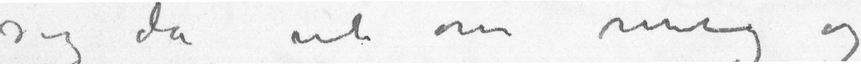sig da ut over mig og
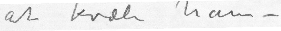at kvæle ham –
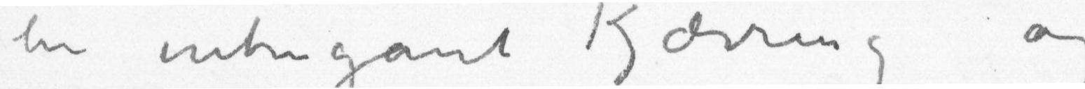en intrigant Kjærring og
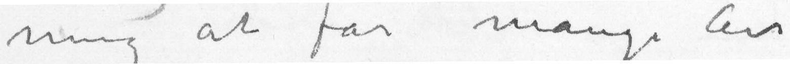mig at for mange År
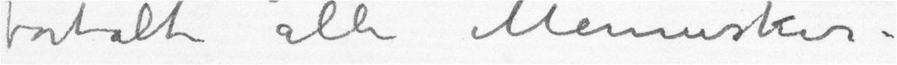fortalte alle Mennesker –
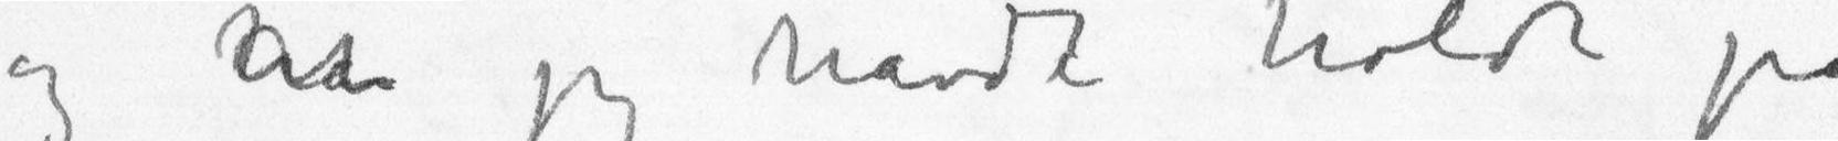og at jeg havde holdt på
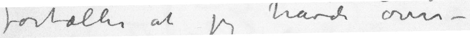fortæller at jeg havde over-
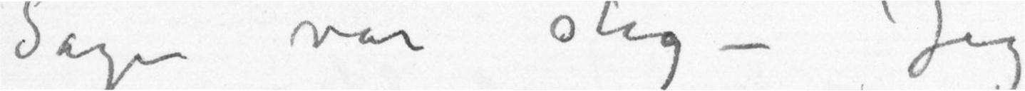Sagen var slig – Jeg
